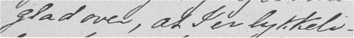glad over, at I er lykkeli-
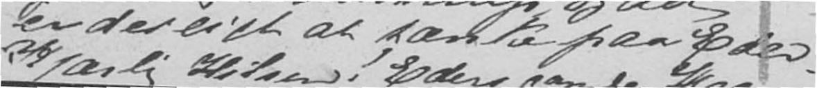er deiligt at tænke paa Eder.
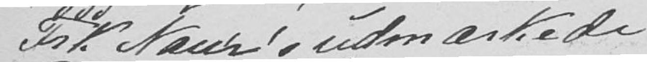Frk Naur's udmærkede
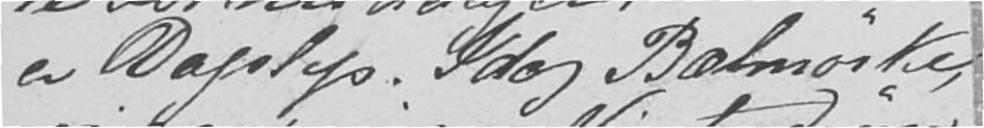er Dagslys. Idag Bælmørke,
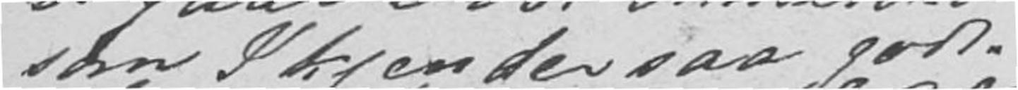som I kjender saa godt.
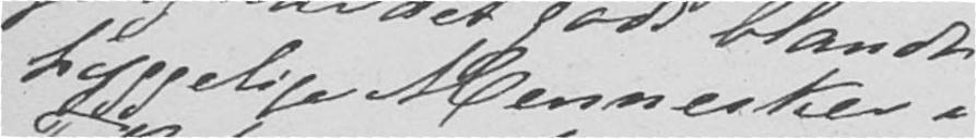hyggelige Mennesker i
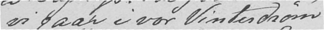vi gaar i vor Vinterdrøm
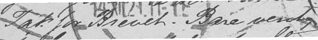Tak for Brevet. Bare vent,
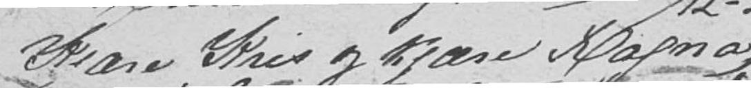Kjære Kris og Kjære Ragna
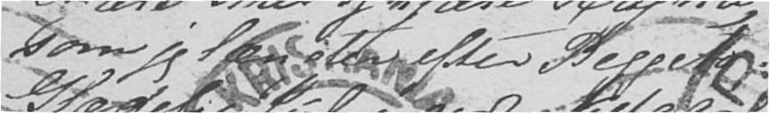som jeg længter efter Beggeto.
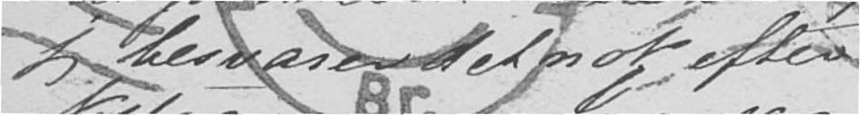jeg besvarer det nok efter
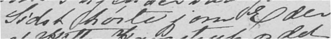Sidst hørte jeg om Eder
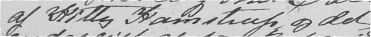af Kitty Kamstrup og det
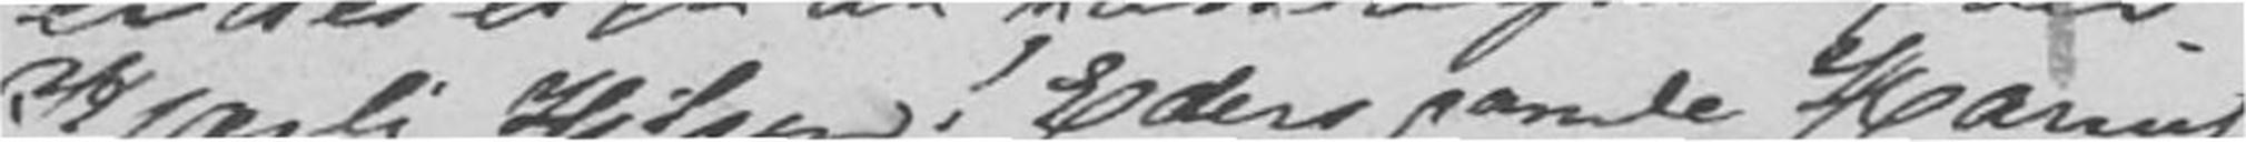Kjærlig Kilsen! Eders gamle Harriet
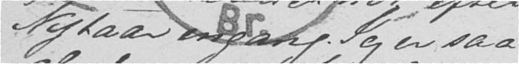Nytaar engang. Jeg er saa
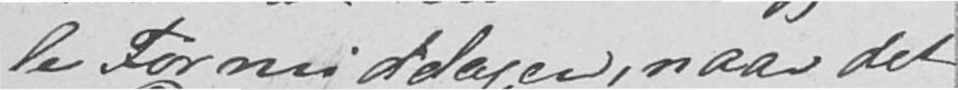le Formiddagen, naar det
